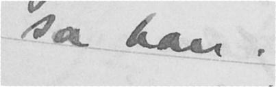sa han.
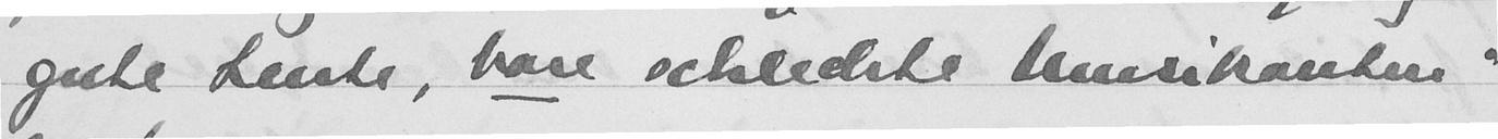gute Leste, bare schlidste Musikanten"
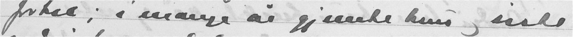fortie; i mange år gjemte hun og viste
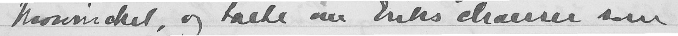Mowinckel, og talte om Eriks chanser som
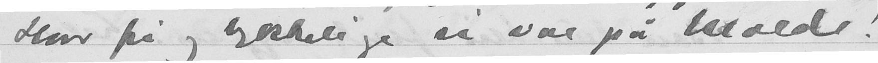Hvor få og lykkelige vi var på Molde!
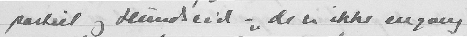partiet og Hundseid - "de er ikke engang
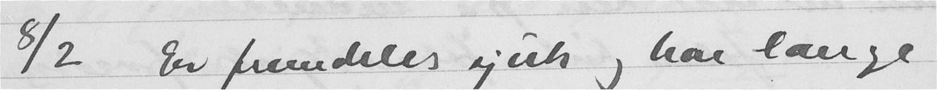8/2 Er fremdeles sjuk og har lange
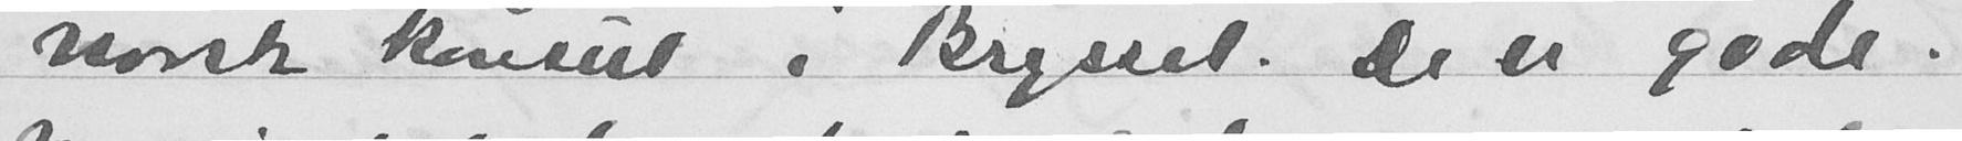norsk konsul i Bergset. De er gode.
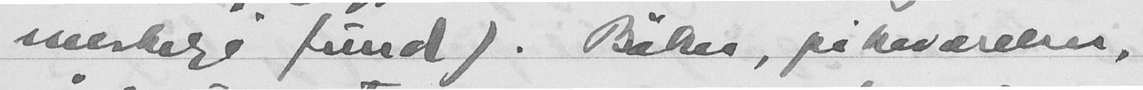merkelige fund). Bøker, pikeværelser,
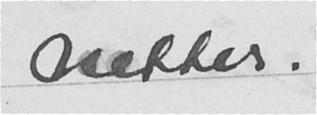netter.
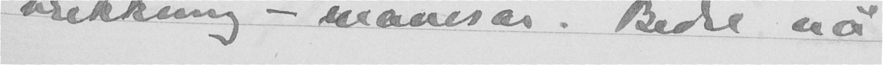brekkning - mavesår. Bedre nå.
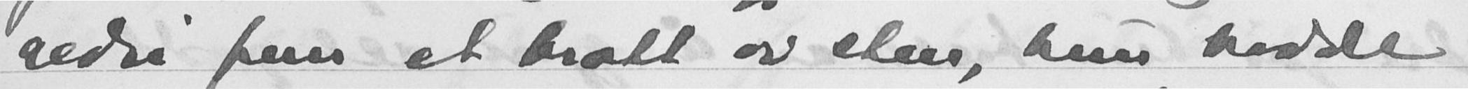andre frem et kratt av sten, hun hadde
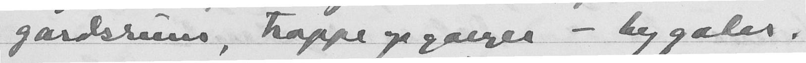gårdsrum, trappeopganger - bygårder.
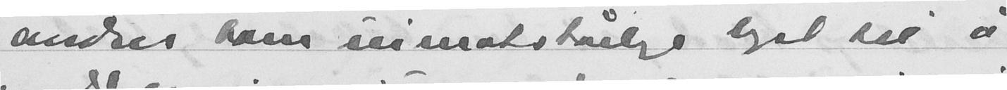andre hans uimotståelige lyst til å
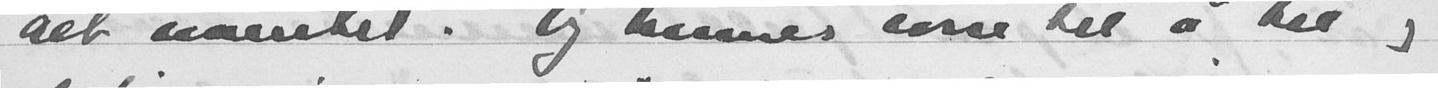reb uventet. Og hennes evne til å tie og
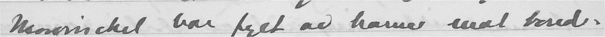Mowinckel har feget av barner mot bonde-
